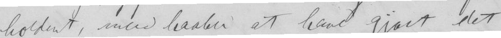holdent, men haaber at have gjort det
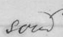som
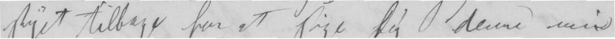skyet tilbage for at sige Dig denne min
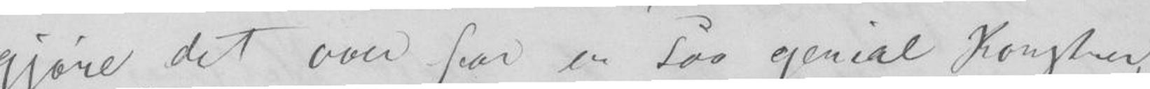gjøre det over for en saa genial Kunstner,
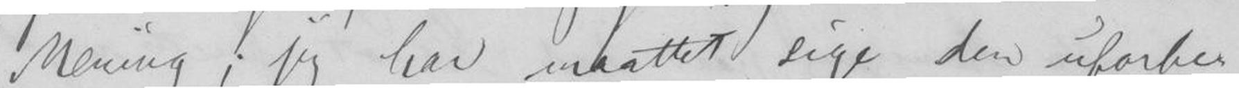Mening; jeg har maattet sige den uforbe-
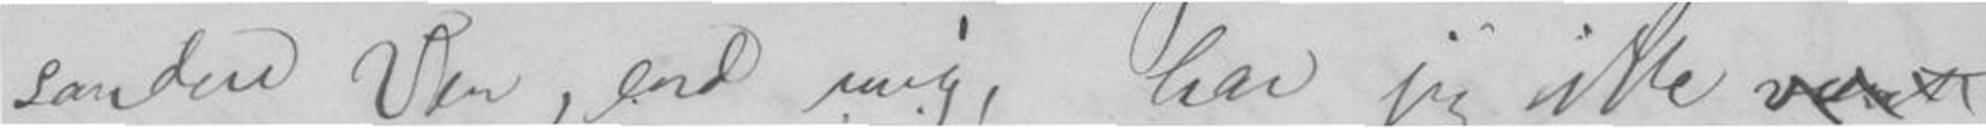sandere Ven, end mig, har jeg ikke været
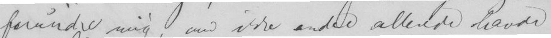forundre mig, om ikke endel allerede havde
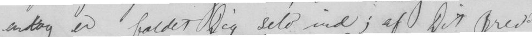endog er faldet Dig selv ind, af Dit Brev
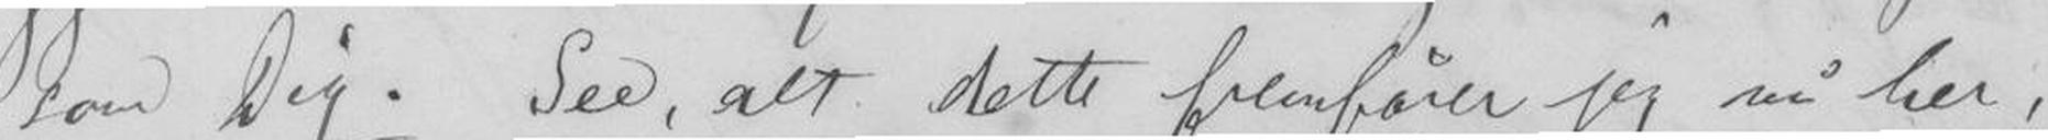som Dig. See, alt dette fremfører jeg nu her,
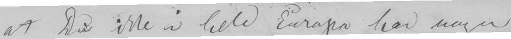at Du ikke i hele Europa har nogen
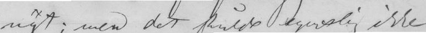nytt; men det skulde egentlig ikke
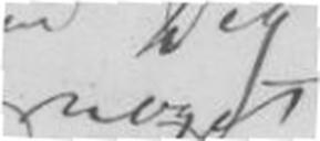noget
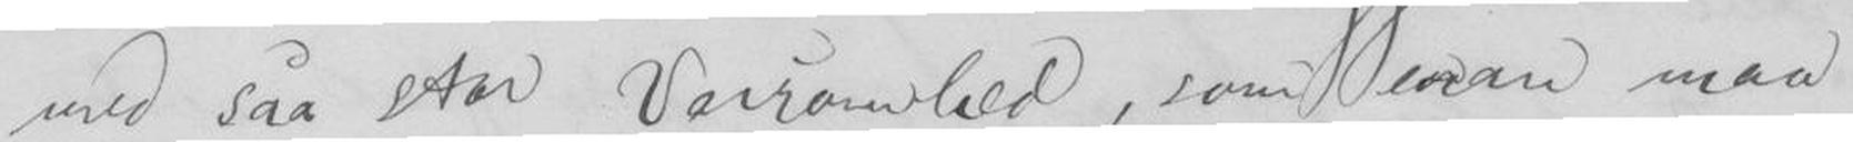med saa stor Varsomhed, som man maa
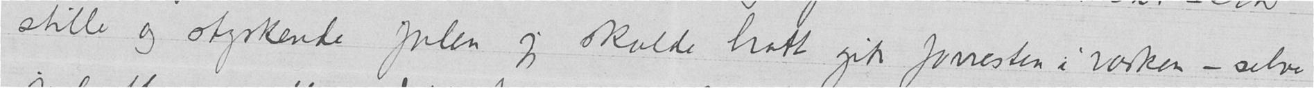stille og styrkende julen jeg skulde hatt gik forresten i vasken - selve
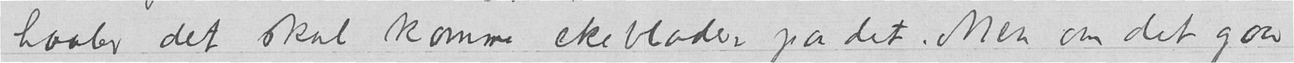haaber det skal komme ekeblader paa det. Men om det gaar
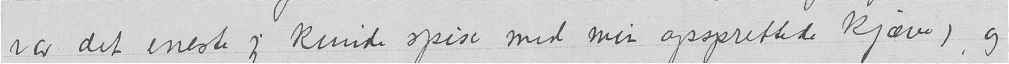var det eneste jeg kunde spise med min opsprettede kjæve), og
